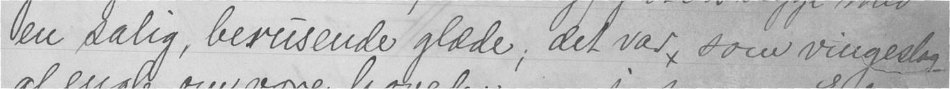ten salig, bereisende glæde; det var, som vingeslag
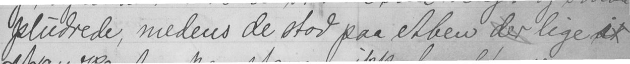pludrede, medens de stod paa et ben der lige i
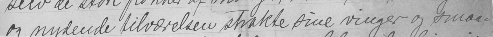og nydende tilværelsen strakte sine vinger og smaa-
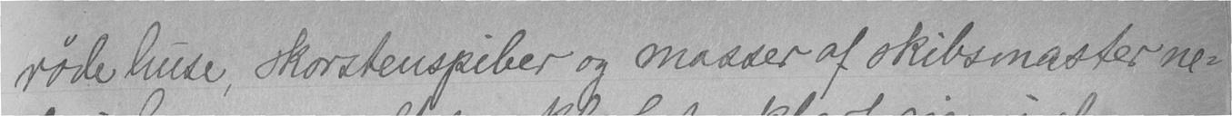røde huse, skorstenspiber og masser af skibsmaster ne-
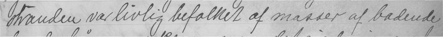stranden var livlig befolket af masser af badende
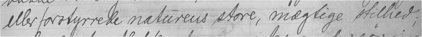eller forstyrrede naturens store, mægtige stilhed;
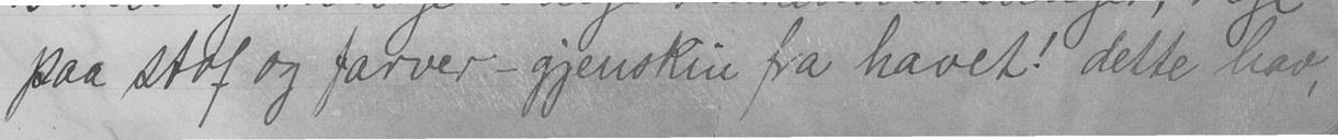paa stof og farver - gjenskin fra havet! dette hav,
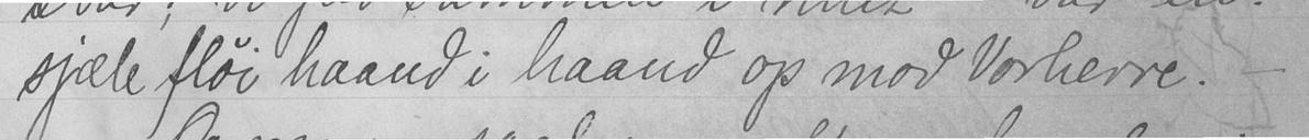sjæle fløi haand i haand op mod Vorherre. -
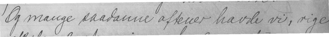Og mange saadanne aftener havde vi, rige
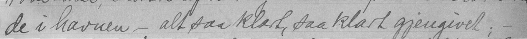de i havnen - alt saa klart, saa klart gjengivet; -
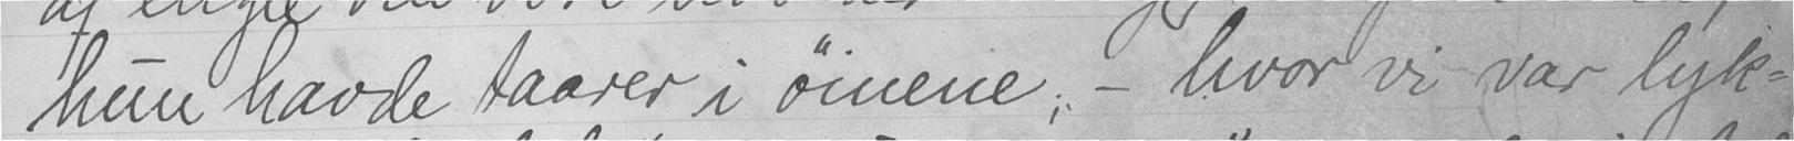hun havde taarer i øinene; - hvor vi var lyk-
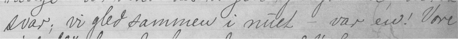svar; vi gled sammen i nuet - var en! Vore
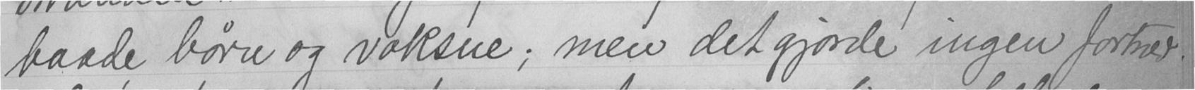baade børn og vaksne; men det gjorde ingen fortred
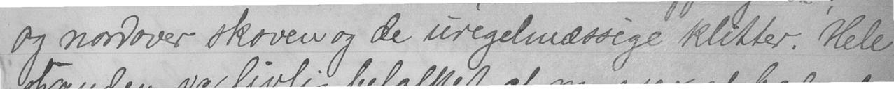og nordover skoven og de uregelmæssige klitter. Hele
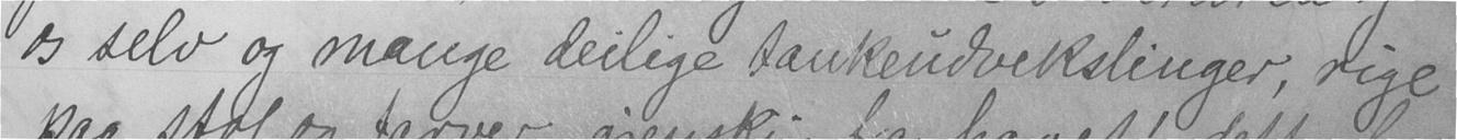os selv og mange deilige tankeudvekslinger, rige
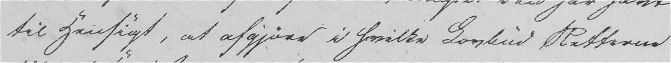til Hensigt, at afgjøre i hvilke Lovbud Retterne
